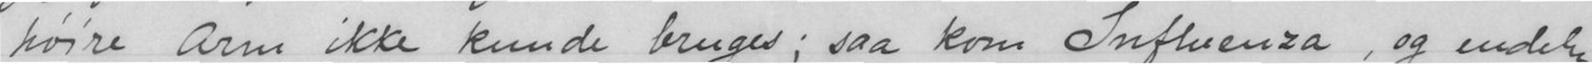høire Arm ikke kunde bruges; saa kom Influenza, og endel
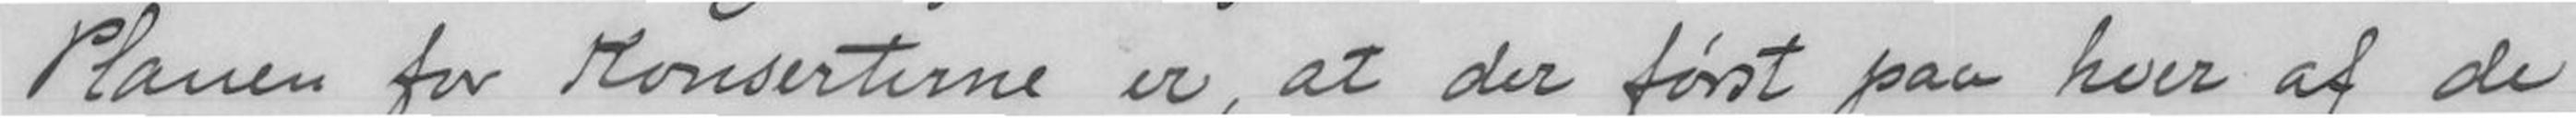Planen for Konserterne er, at der først paa hver af de
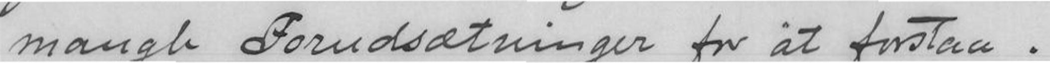mangle Forudsætninger for at forstaa.
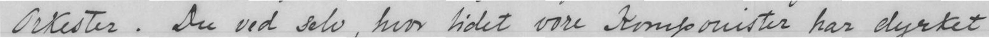Orkester. Du ved selv, hvor lidet vore Komponister har dyrket
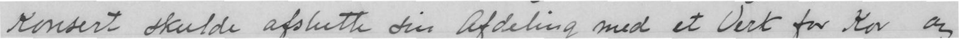Konsert skulde afslutte sin Afdeling med et Verk for Kor og
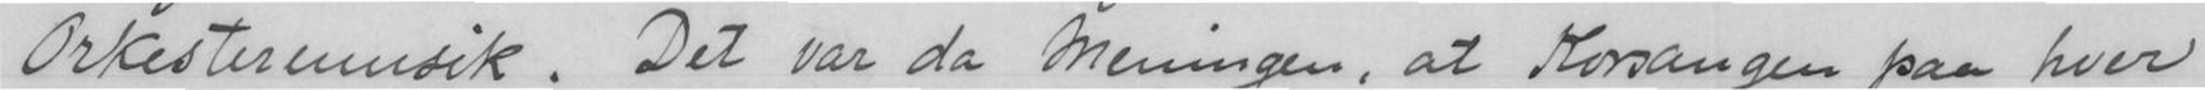Orkestermusik. Det var da Meningen, at Korsangen paa hver
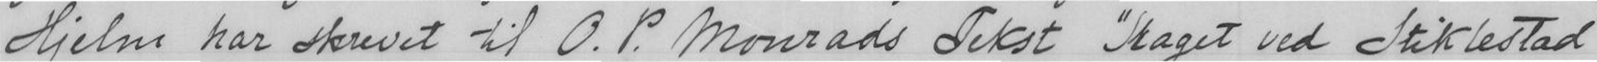Hjelm har skrevet til O.P.Monrads Tekst "Skaget ved Stiklestad
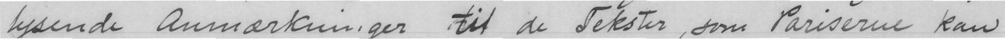lysende Anmærkninger til de Tekster, som Pariserne kan
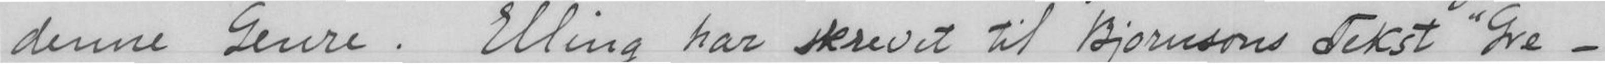denne Gener Elling har skrevet til Bjørnsons Tekst "Gre-
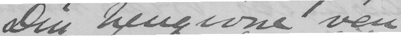Din hengivne ven
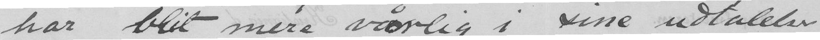har blit mere vårlig i sine udtalelser
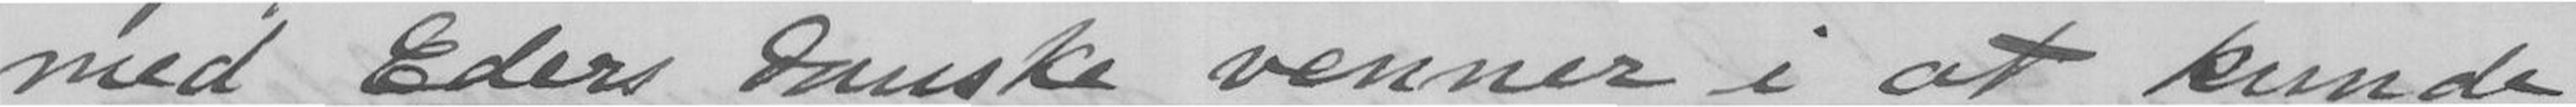med Eders danske venner i at kunde
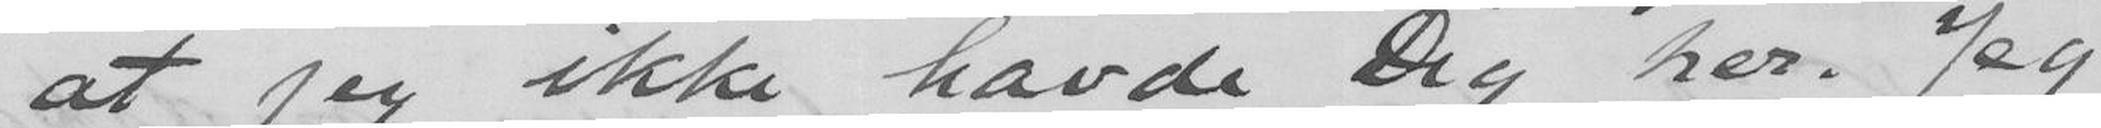at jeg ikke havde Dig her. Jeg
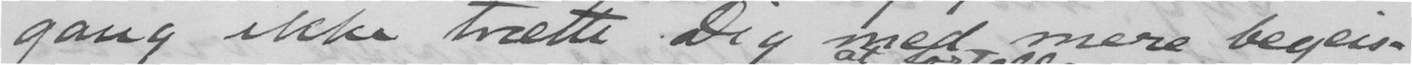gang ikke trette Dig med mere begeis-
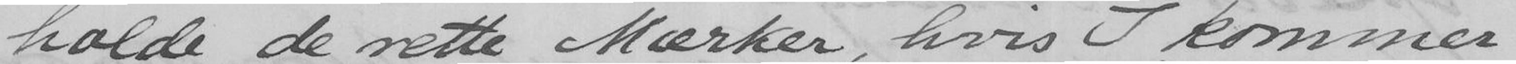holde de rette Mærker, hvis I kommer
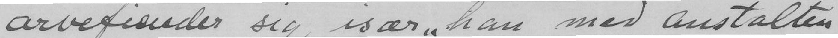arvefiender sig, især han med anstalten
